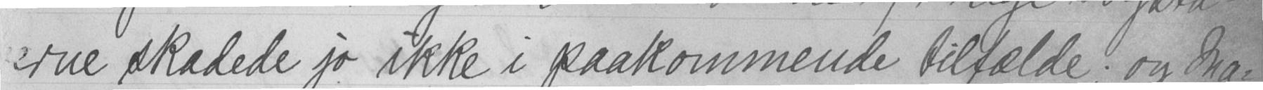erne skadede jo ikke i paakommende tilfælde; og Ma-
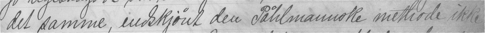det samme, endskjønt den Påhlmannske methode ikke
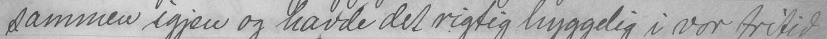sammen igjen og havde det rigtig hyggelig i vor fritid
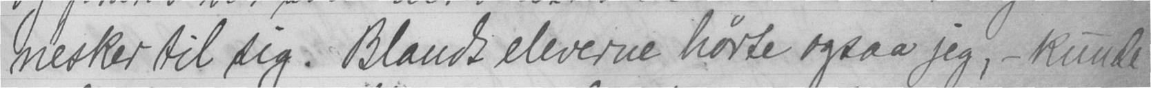nesker til sig. Blandt eleverne hørte ogsaa jeg, -  kunde
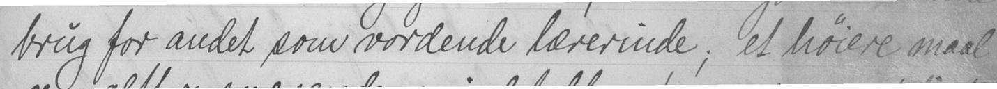brug for andet som vordende lærerinde; et høiere maal
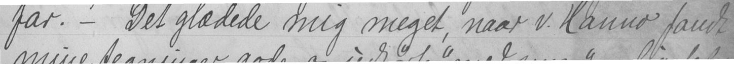far. - Det glædede mig meget, naar V. Hanno fandt
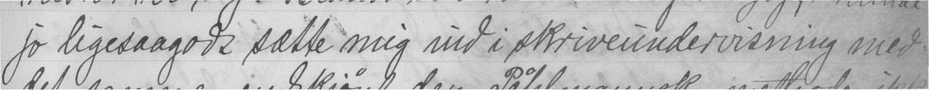jo ligesaagodt sætte mig ind i skriveundervisning med
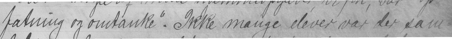fatning og omtanke". Ikke mange elever var der sam-
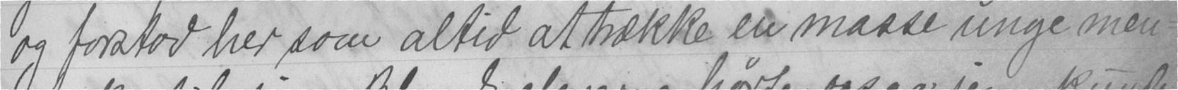og forstod her som altid at trække en masse unge men-
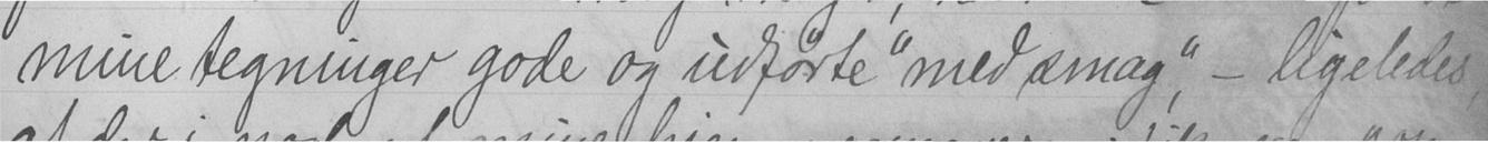mine tegninger gode og udførte "med smag", - ligeledes,
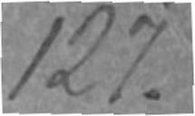127.
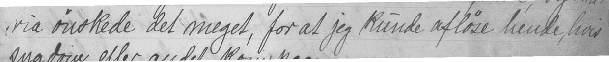ria ønskede det meget, for at jeg kunde afløse hende, hvis
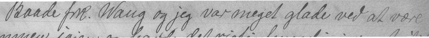Baade frk. Wang og jeg var meget glade ved at være
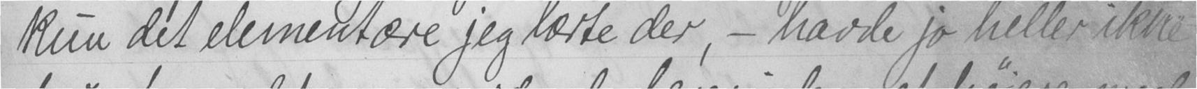kun det elementære jeg lærte der, - havde jo heller ikke
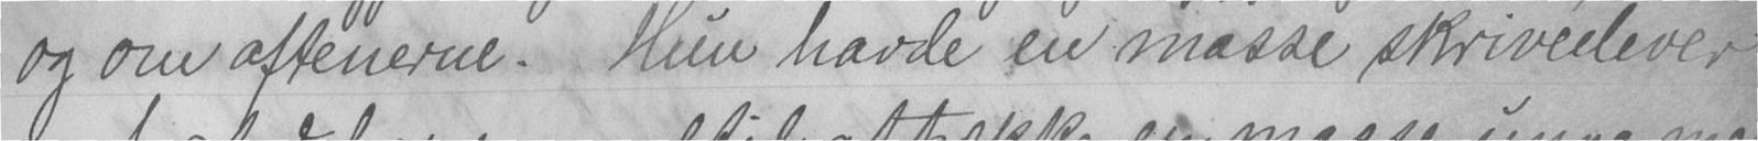og om aftenerne. Hun havde en masse skriveelever
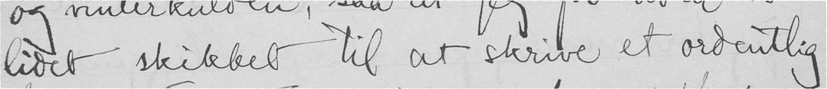lidet skikket til at skrive et ordentlig
